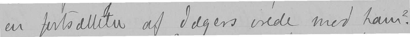en fortsættelse af Jægers vrede med ham?
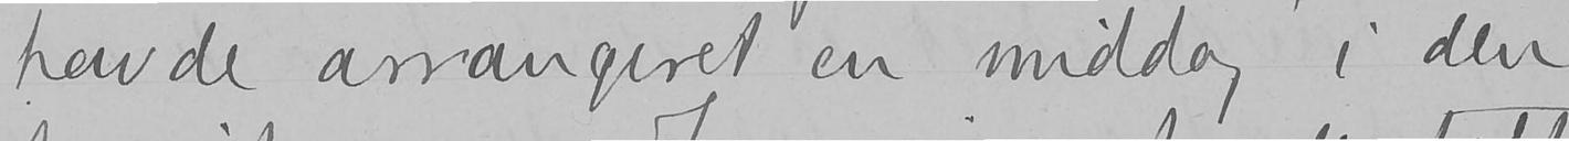havde arrangeret en middag i den
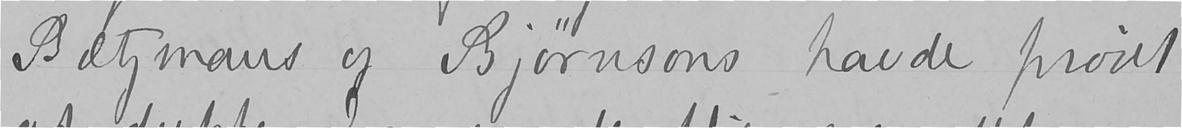Bætzmans og Bjørnsons havde prøvet
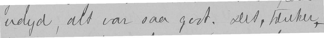udyd, alt var saa godt. Det, tænker
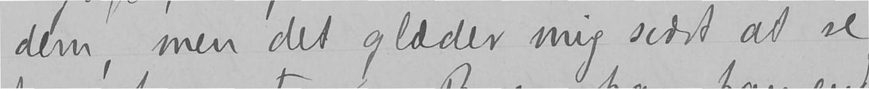dem, men det glæder mig svært at se,
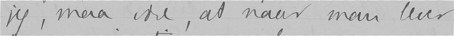jeg, maa være, at naar man lever
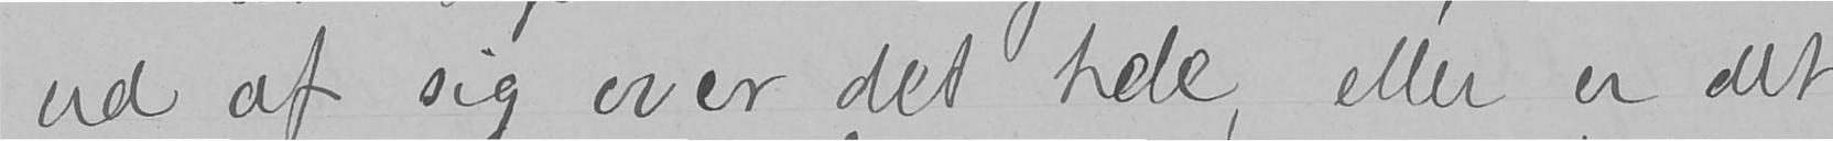ud af sig over det hele, eller er det
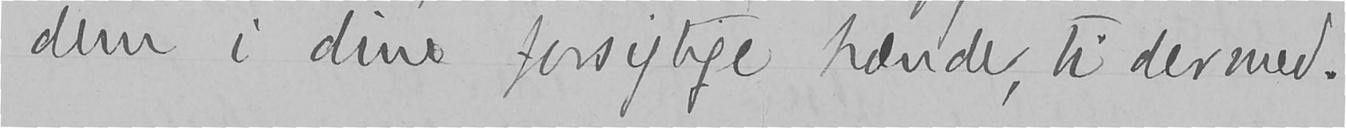dem i dine forsigtige hænder, ti dermed.
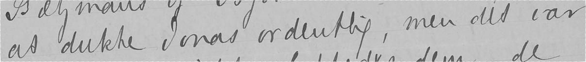at dukke Jonas ordentlig, men det var
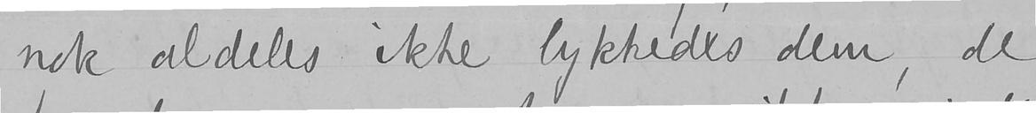nok aldeles ikke lykkedes dem, de
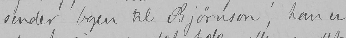sender bogen til Bjørnson, han er
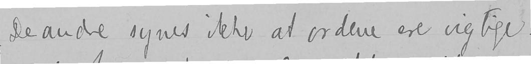De andre synes ikke at ordene ere vigtige.
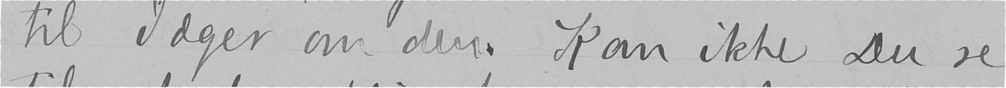til Jæger om den. Kan ikke Du se
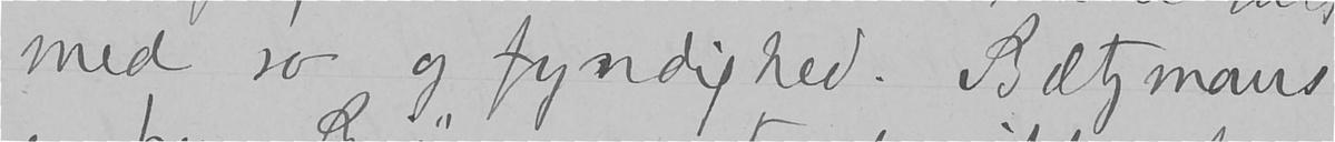med ro og fyndighed. Bætzmans
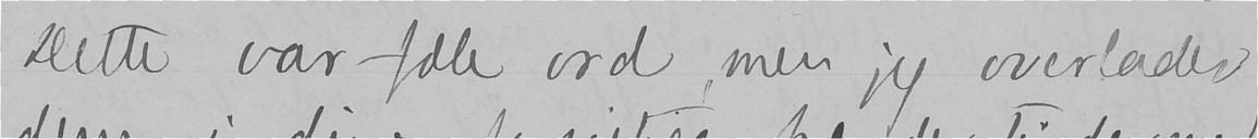Dette var fæle ord, men jeg overlader
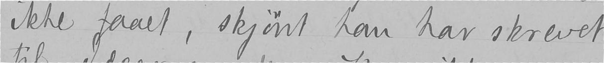ikke faaet, skjønt han har skrevet
